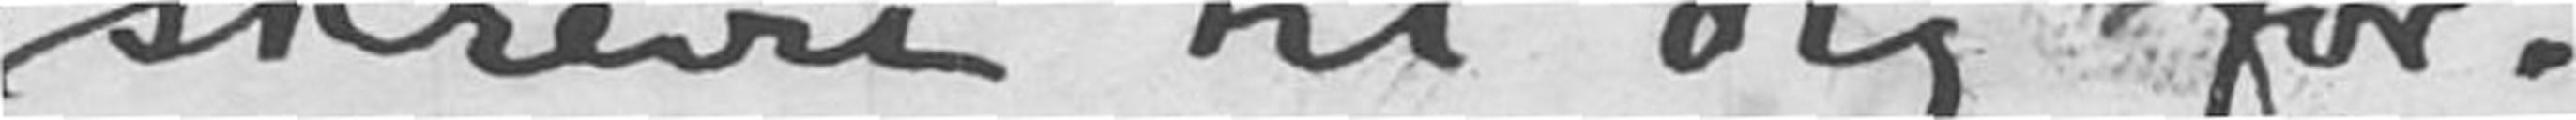skrevet til dig før.
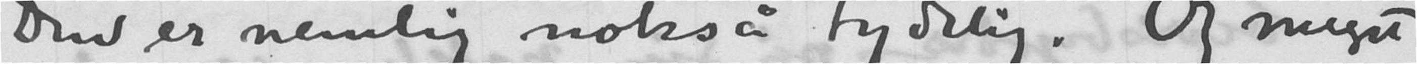Den er nemlig nokså tydelig. Og meget
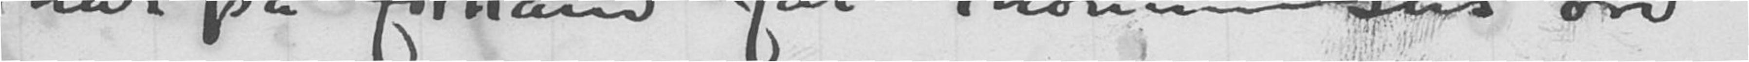har på forhånd fåt Thommessens ord
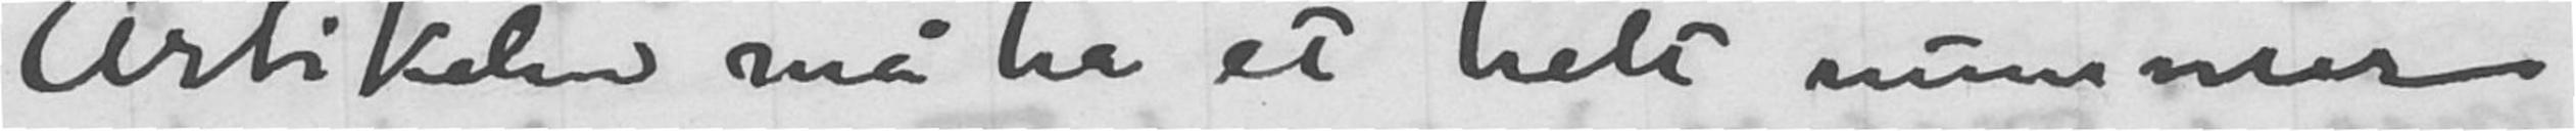Artikelen må ha et helt nummer -
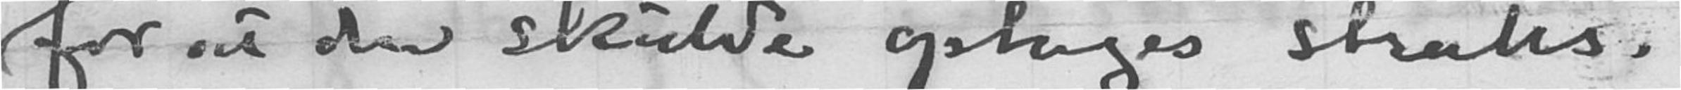for at den skulde optages straks.
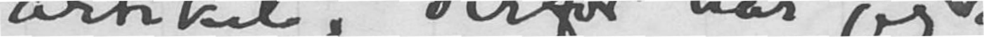artikel, derfor har jeg
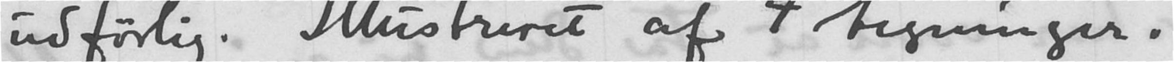udførlig. Illustreret af 4 tegninger.
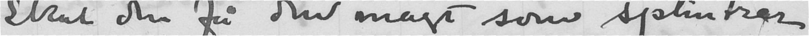Skal den få den magt som splintrer
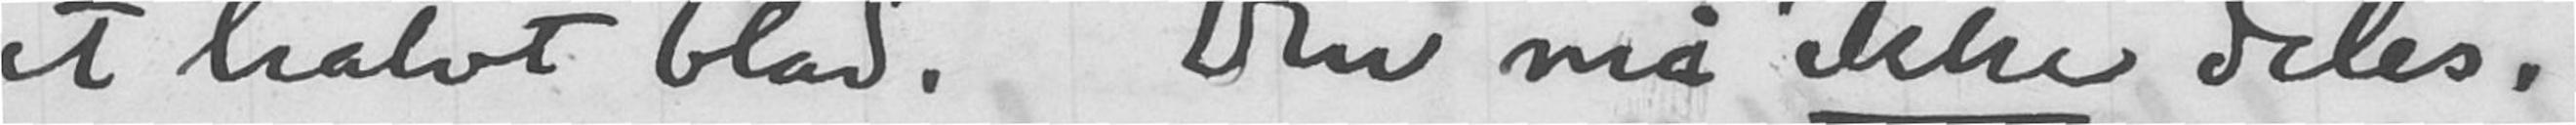et halvt blad. Den må ikke deles,
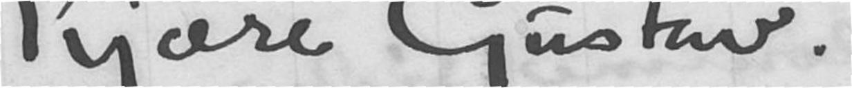Kjære Gustav.
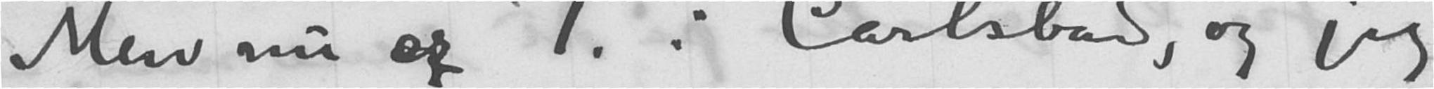Men nu er T. i Carlsbad, og jeg
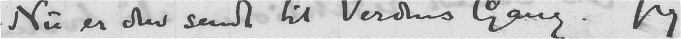Nu er den sendt til "Verdens Gang". Jeg
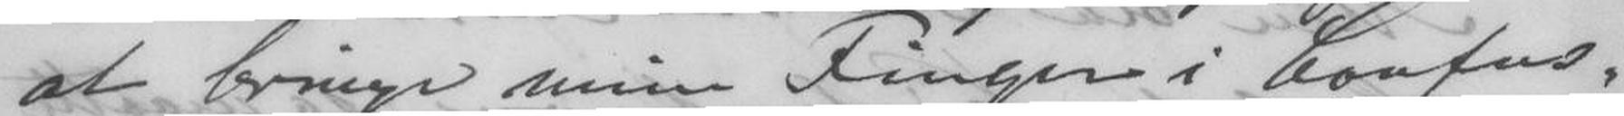at bringe min Finger i Confus-
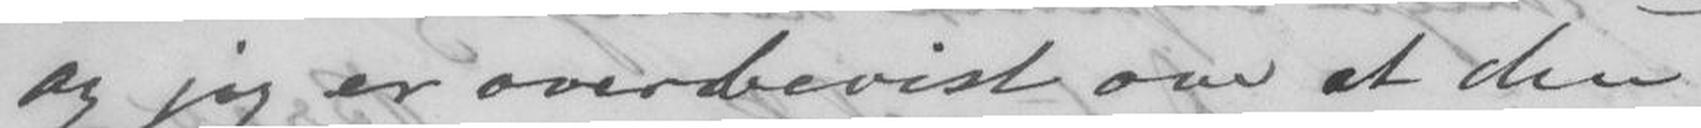og jeg er overbevist om at den
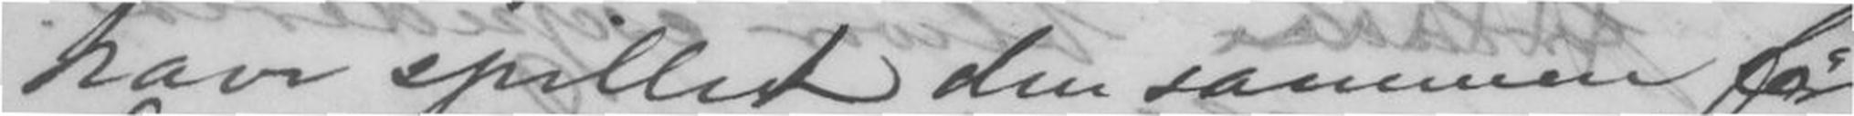have spilled den sammen før
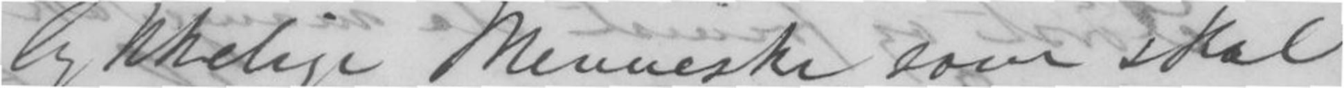lykkelige Menneske, som skal
